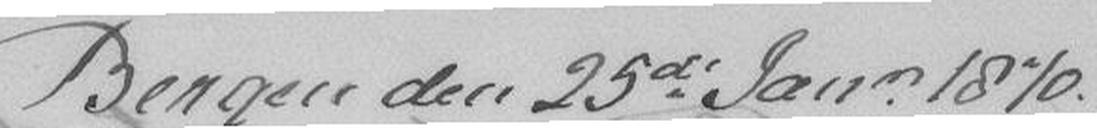Bergen den 25deJanr 1870.
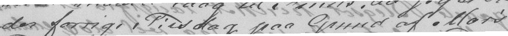der forrige Tirsdag paa Grund af Mor's
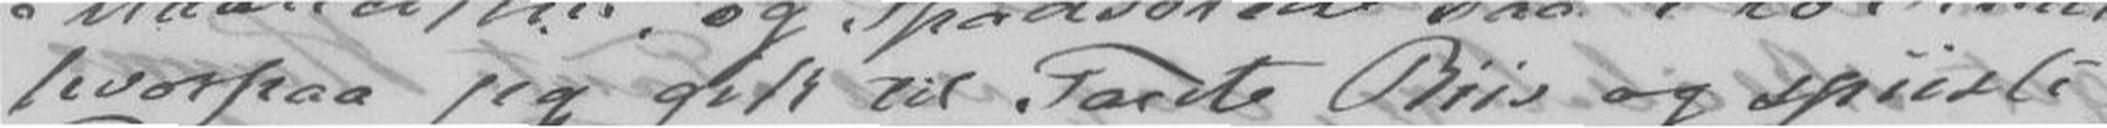hvorpaa jeg gik til Tante Riis og spiiste
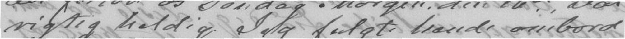rigtig heldig. Jeg fulgte hende ombord
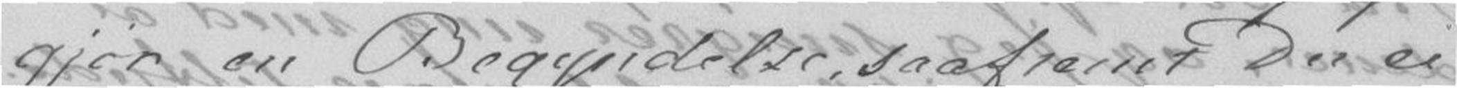gjør en Begyndelse, saafremt Du ei
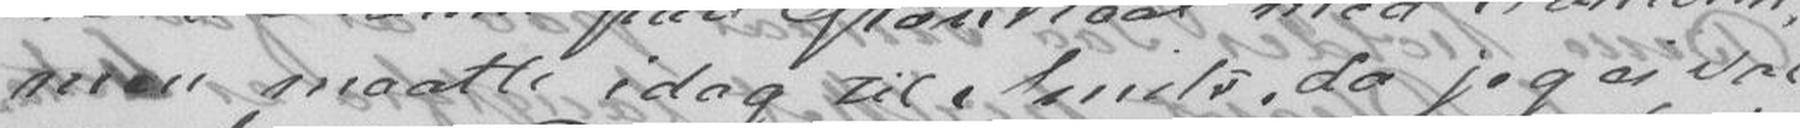men maatte idag til Smits, da jeg ei var
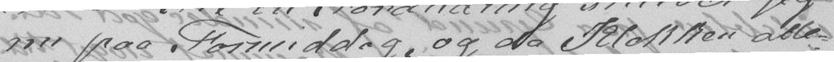nu paa Formiddag, og da klokken alle-
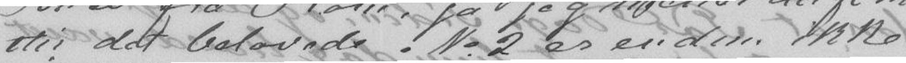thi det belovede N.2 er endnu ikke
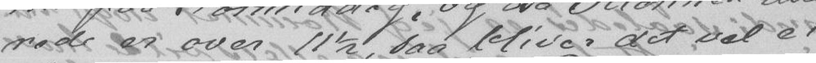rede er over 11½, saa bliver det vel ei
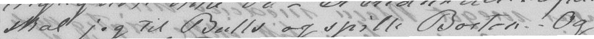skal jeg til Bulls og spille Boston. Og
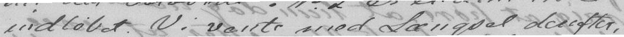indløbet. Vi vente med Længsel derefter,
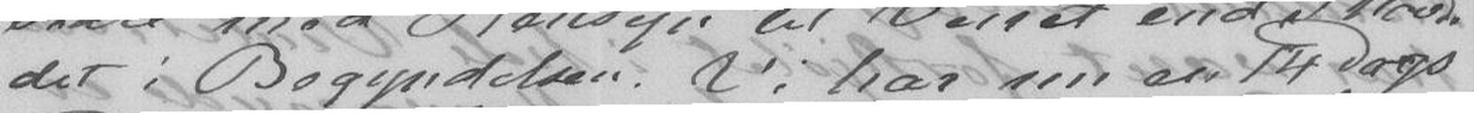det i Begyndelsen. Vi har nu en 14 Dags
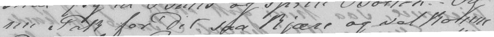nu Tak for Dit saa kjære og velkomne
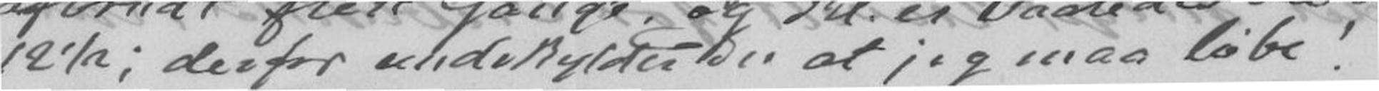12½; derfor undskylder Du at jeg maa løbe!
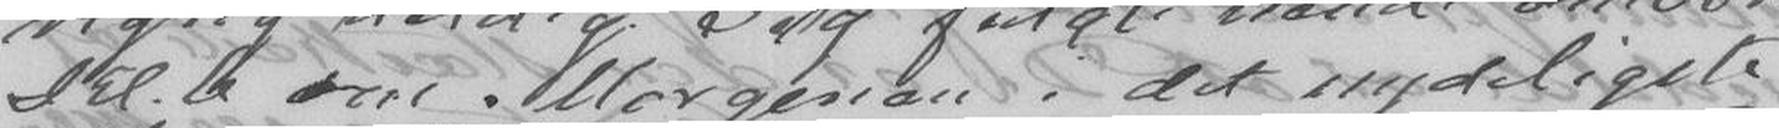Kl.6 om Morgenen i det nydeligste
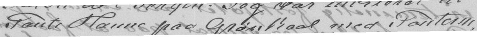Tante Hanne paa Grønkaal med Tanterne,
